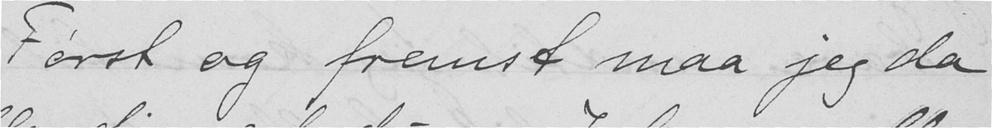Først og fremst maa jeg da
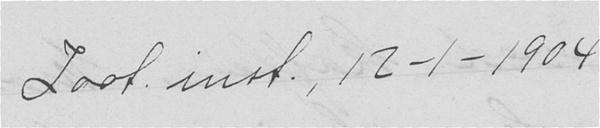Zool. inst., 12-1-1904
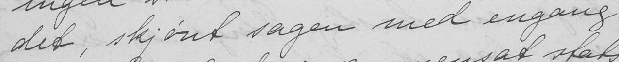det, skjønt sagen med engang
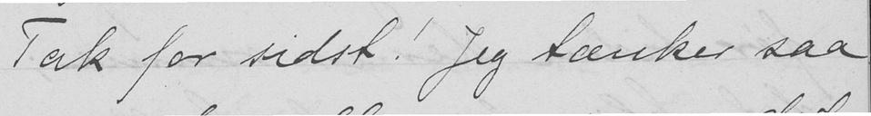Tak for sidst! Jeg tænker saa
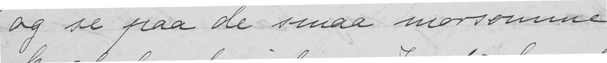og se paa de smaa morsomme
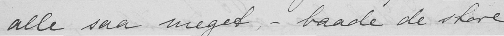alle saa meget, - baade de store
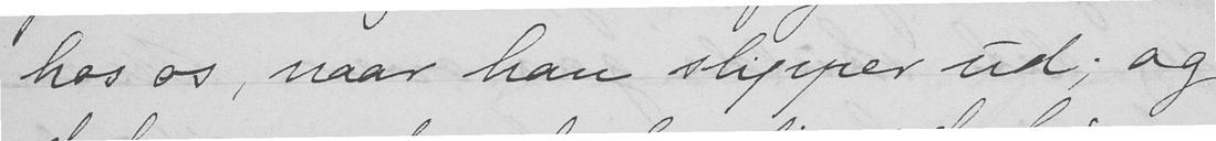hos os, naar han slipper ud; og
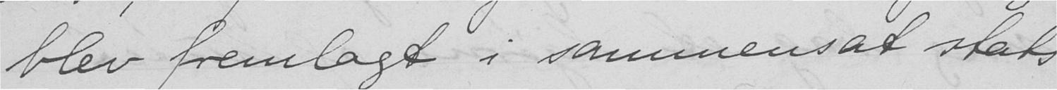blev fremlagt i sammensat stats-
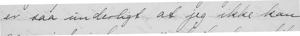er saa underligt, at jeg ikke kan
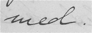med.
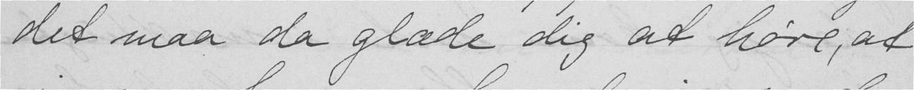det maa da glæde dig at høre, at
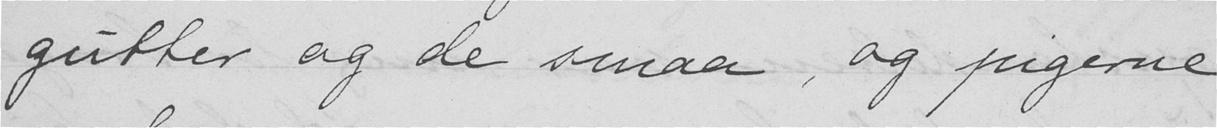gutter og de smaa, og pigerne
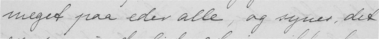meget paa eder alle, og synes, det
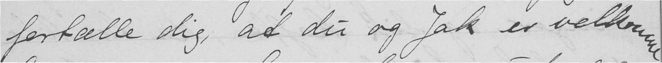fortælle dig at du og Jak er velkommen
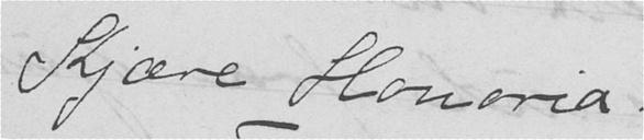Kjære Honoria!
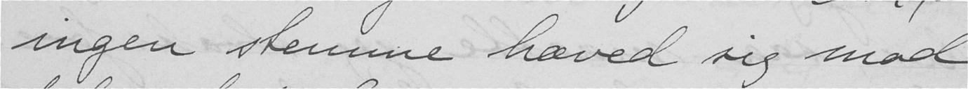ingen stemme hæved sig mod
